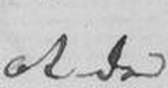at da
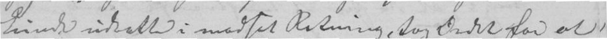kunde udrette i modsat Retning, tog Ordet for at
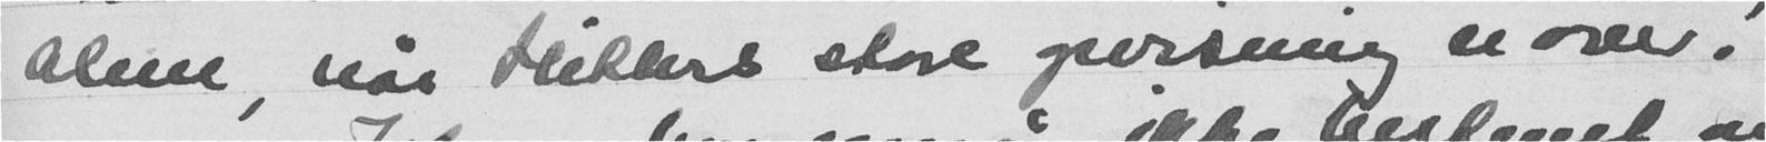alene, når Hitlers store opvisning er over!
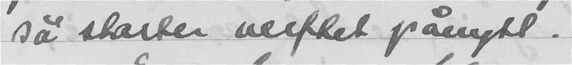så starter verftet pånytt.
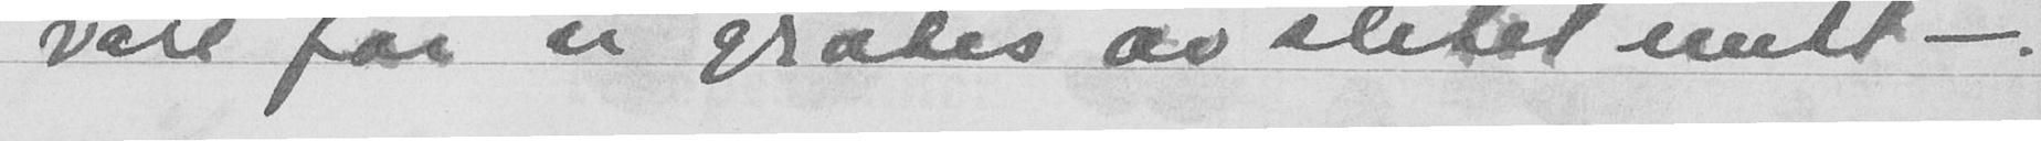våre får vi gratis av slitet mitt -.
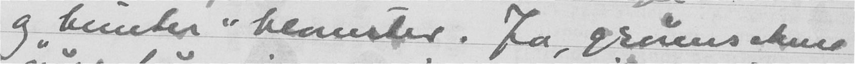og "bunter" blomster. Ja, grånens skue
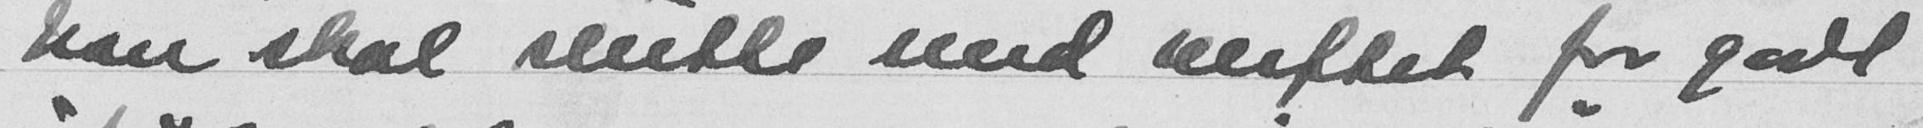han skal slutte med verftet for godt
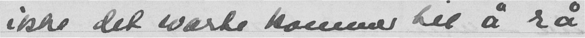ikke det svarte kommer til å rå
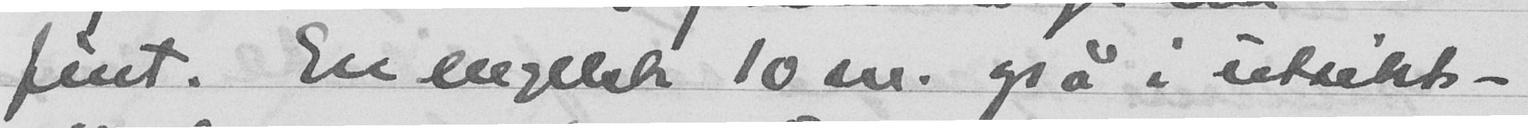fint. En engelsk 10 m. også i utsikt -
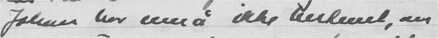Johans har ennå ikke bestemt, om
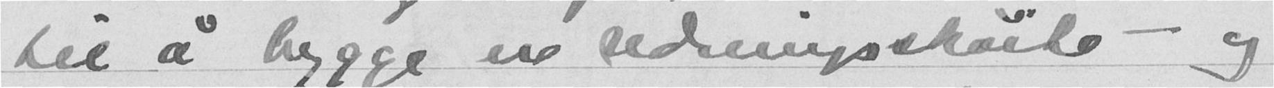til å bygge en rednigsskute og
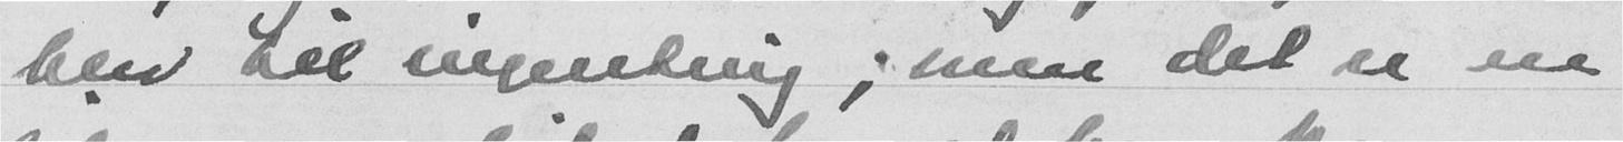blir vel ingenting; men det er en
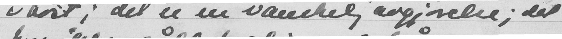i høst; det er en vanskelig avgjørelse; det
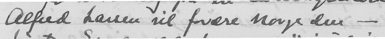Alfred Larsen vil forære Norge den -
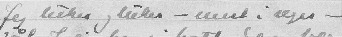Jeg luker og luker - mest i seger -
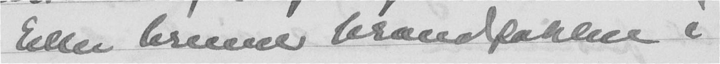Eller brenner brandfaklene i
